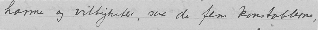harme og vittigheter, saa de fem konstablerne,
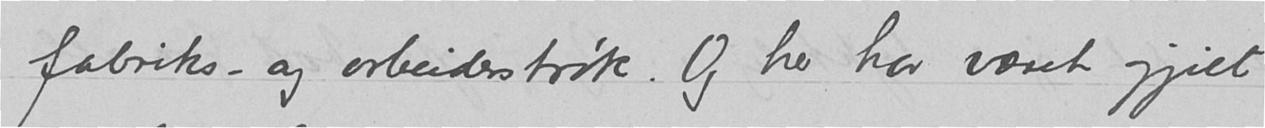fabriks-og arbeiderstrøk. Og her har været gjilt
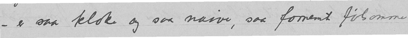- er saa kloke og saa naive, saa fornemt følsomme
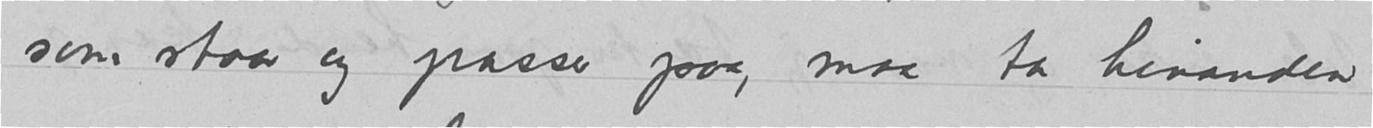som staar og passer paa, maa ta hinanden
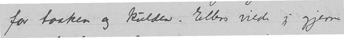for taaken og kulden. Ellers vilde jeg gjerne
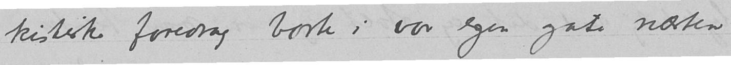kistiske foredrag borte i vor egen gate næsten
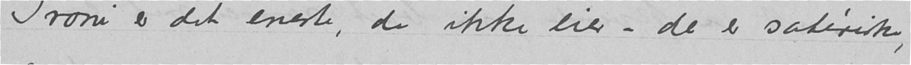Ironi er det eneste, de ikke eier – de er satiriske,
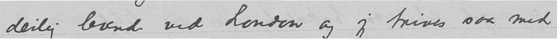deilig levende ved London og jeg trives saa med
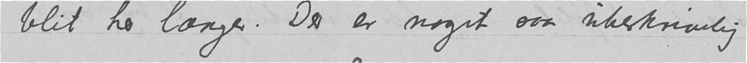blit her længer. Der er noget saa ubeskrivelig
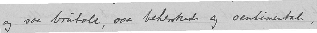og saa brutale, saa beherskede og sentimentale,
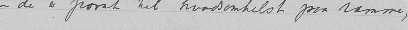– de er parat til hvadsomhelst paa ramme,
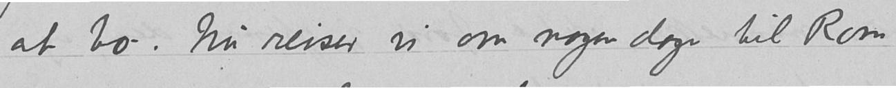at bo. Nu reiser vi om nogen dage til Rom
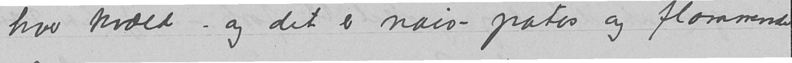hver kvæld - og det er nais - patos og flammende
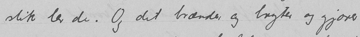slik ler de. Og det brænder og bryter og gjærer
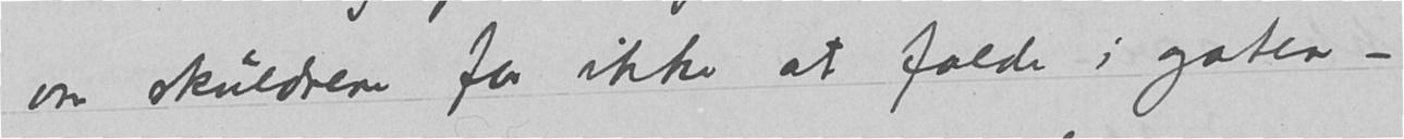om skuldrene for ikke at falde i gaten -
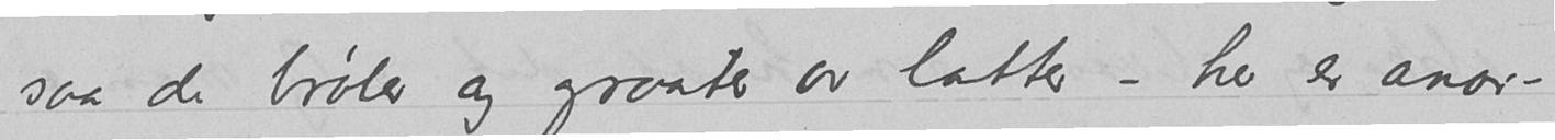saa de brøler og graater av latter – her er anar-
